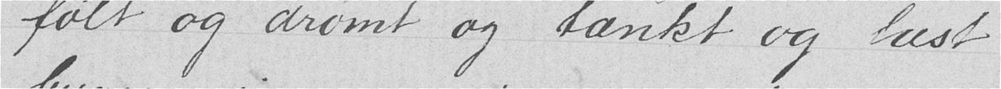følt og drømt og tænkt og læst
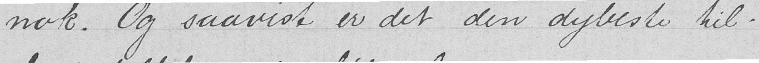nok. Og saavist er det den dybeste til-
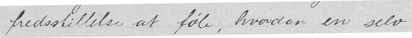fredsstillelse at føle, hvordan en selv
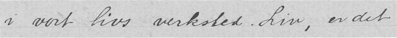i vort livs verksted. Liv, er det
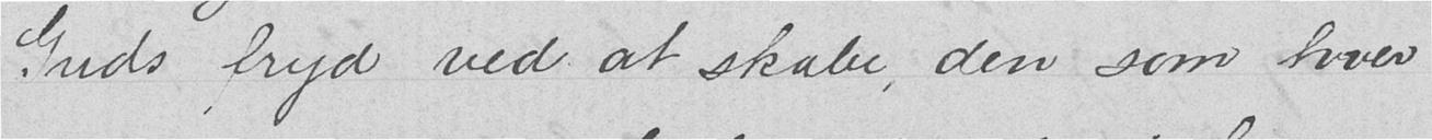Guds fryd ved at skabe, den som hver
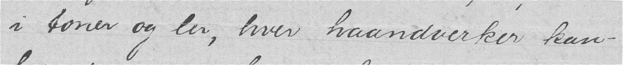i toner og ler, hver haandværker kan-
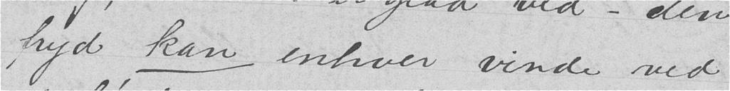fryd kan enhver vinde ved
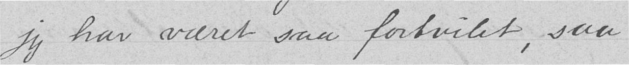jeg har været saa fortvilet, saa
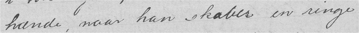hænde, naar han skaber en ringe
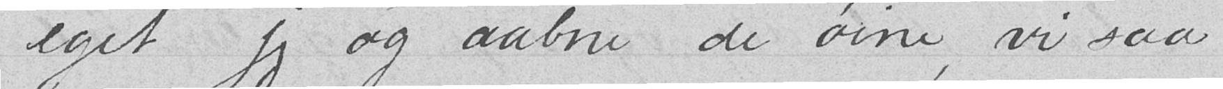eget jeg og aabne de øine, vi saa
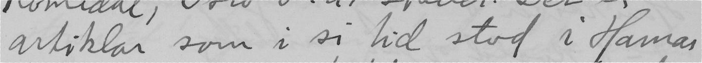artiklar som i si tid stod i Hamar
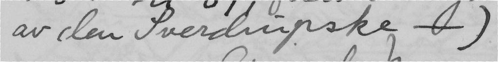av den Sverdrupske –)
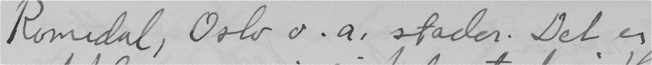Romsdal, Oslo o.a. stader. Det er
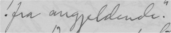fra angjeldende".
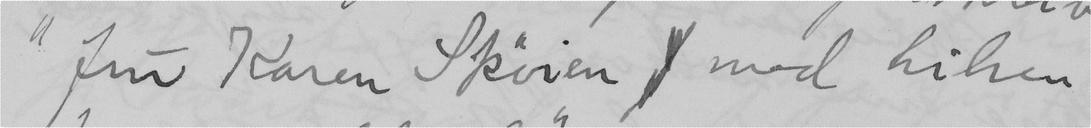"fru Karen Sköien  med hilsen
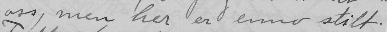oss, men her er enno stilt.
